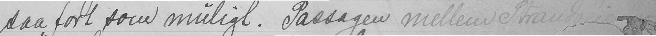saa fort som muligt. Passagen mellem Strand
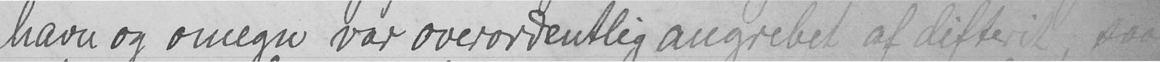havn og omegn var overordentlig angrebet af difteril, saa
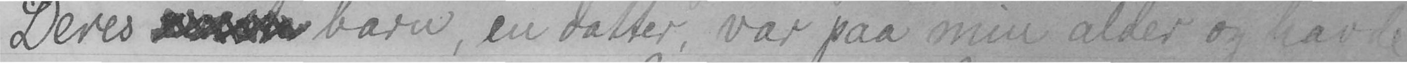Deres barn, en datter, var paa min alder og havde
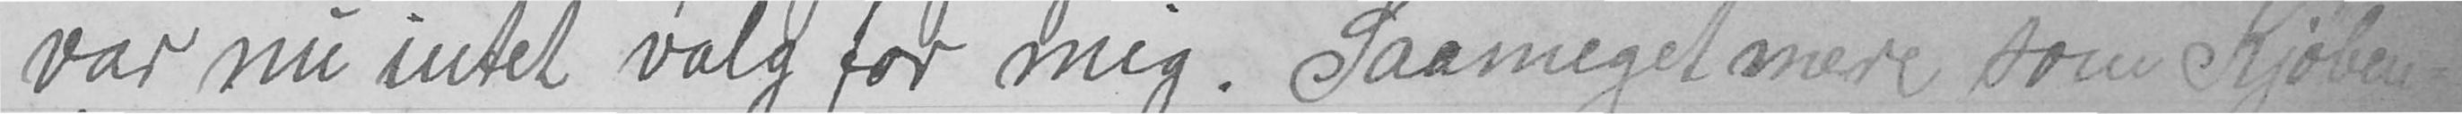var nu intet valg for mig. Saameget mere som Kjøben-
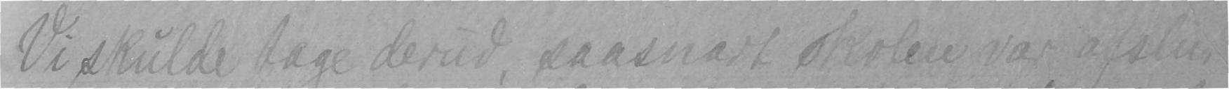Viskulde tage derind, saasnart skolen var afslutted.
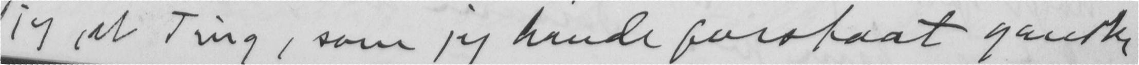jeg at Ting, som jeg havde forstaat ganske
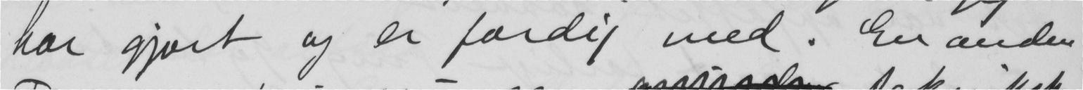har gjort og er færdig med. En anden
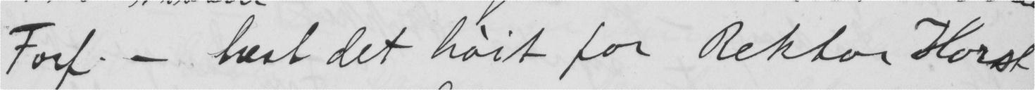Forf. - læst det høit for Rektor Horst
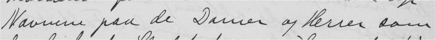Navnene paa de Damer og Herrer som
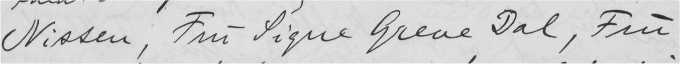Nissen, Fru Signe Greve Dal, Fru
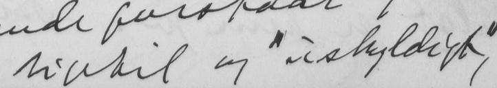rivtil og "uskyldigt",
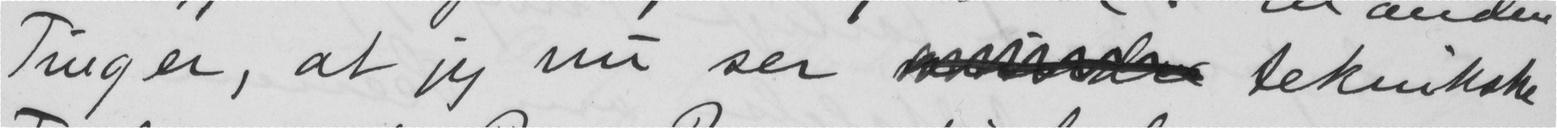Tinger, at jeg nu ser  teknikske
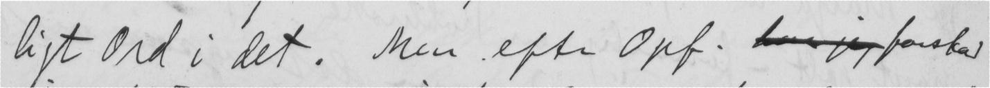ligt Ord i det. Men efter Opf.  forstod
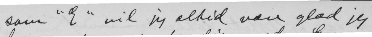som "E" vil jeg altid være glæd jeg
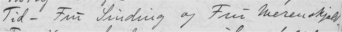Tid - Fru Sinding og Fru Werenskjold
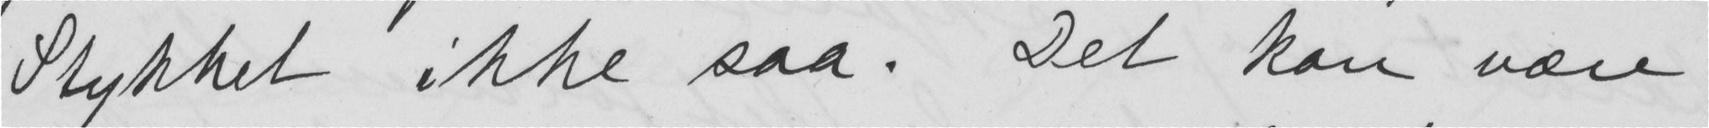Stykket ikke saa. Det kan være
